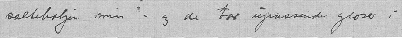saltebaljen min" – og de tar upassende gloser i
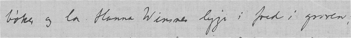bøker og la Hanna Winsnes ligge i fred i graven,
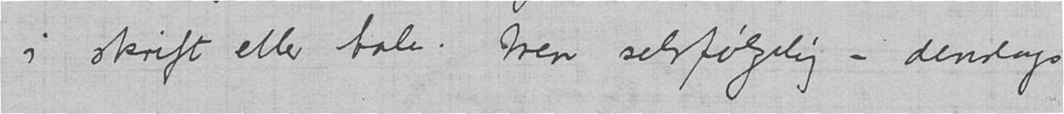i skrift eller tale. Men selvfølgelig - denslags
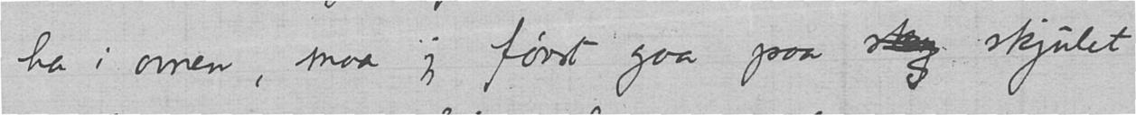ha i ovnen, maa jeg først gaa paa meg skjulet
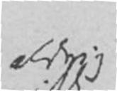aldrig
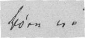Børn ere
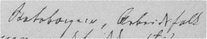Statsborgere, Arbeidsfolk
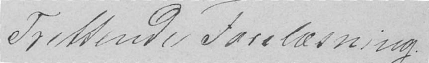Trettende Forelæsning.
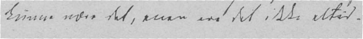kunne være det, men er det ikke altid.
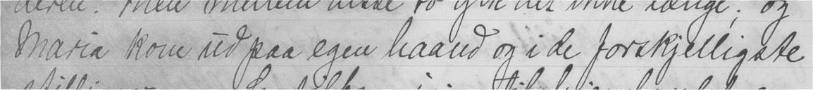Maria kom ud paa egen haand og i de forskjelligste
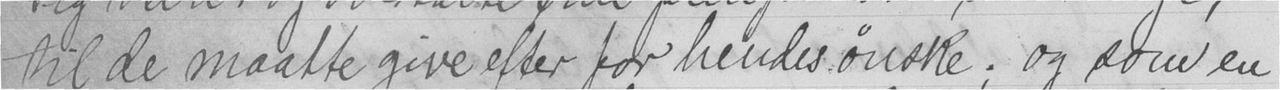til de maatte give efter for hendes ønske; og som en
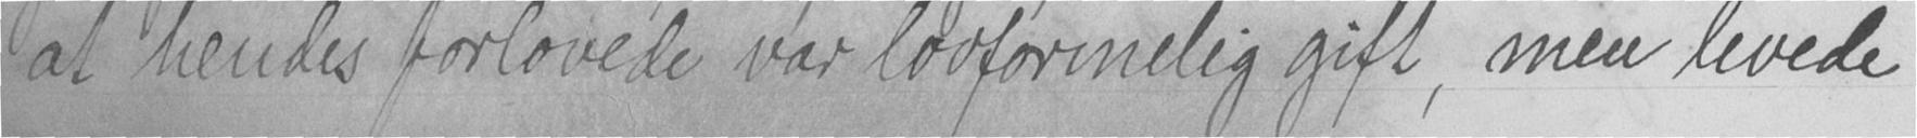at hendes forlovede var lovformelig gift, men levede
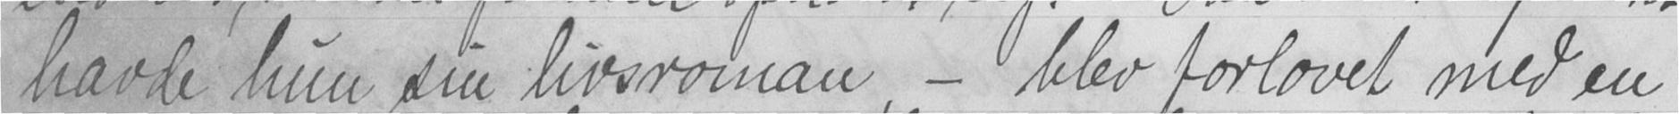havde hun sin livsroman, - blev forlovet med en
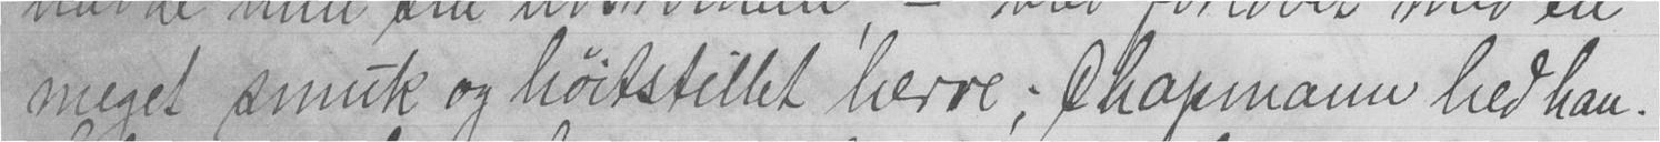meget smuk og høitstillet herre; Chapmann hed han.
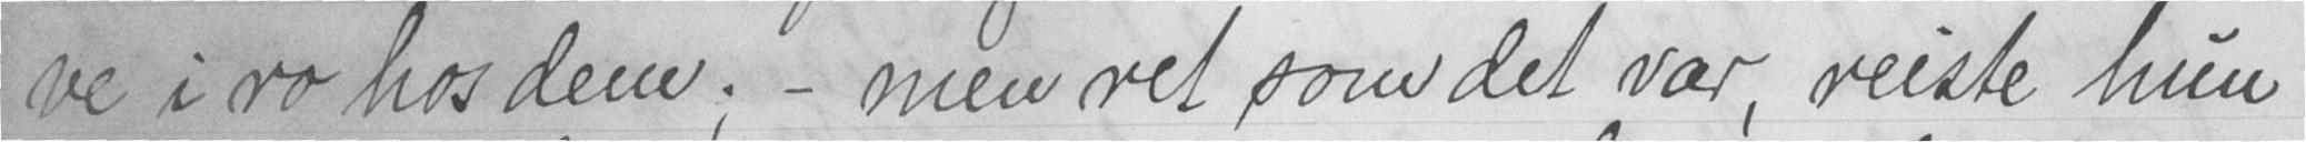ve i ro hos dem; - men ret som det var, reiste hun
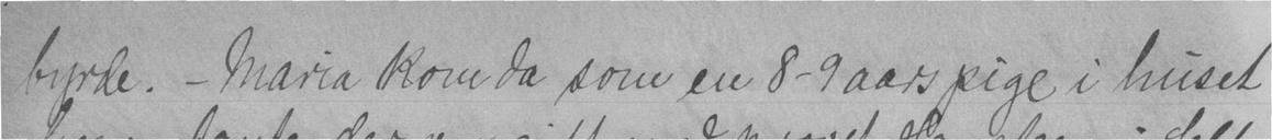byrde. - Maria kom da som en 8-9 aarspige i huset
